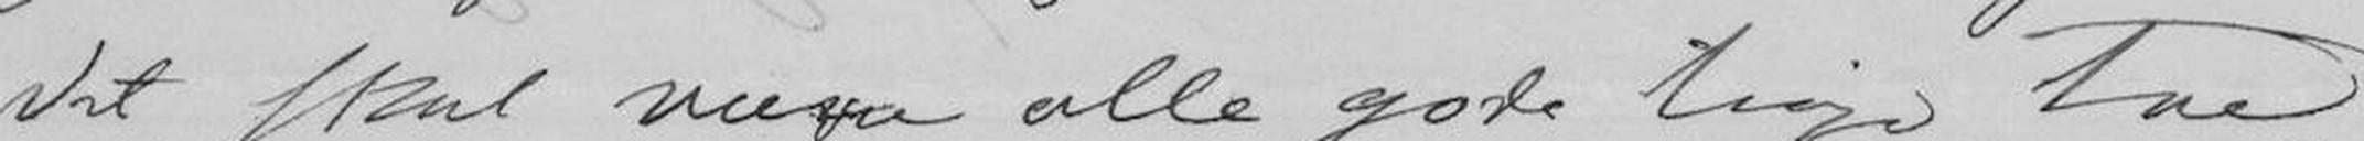det skal væra alle gode tinge tre,
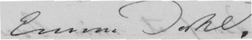Emma Dahl
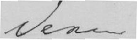Deres
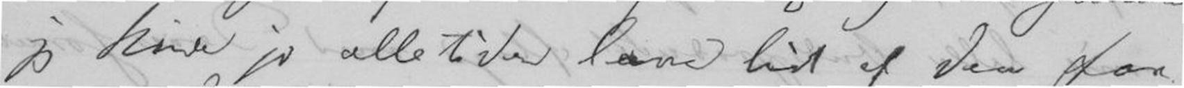jag kunde jo alle tider lave lid af den saa
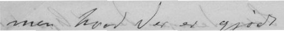men hvad der er gjødt
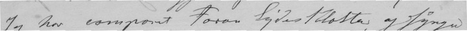Jeg har componert Foran Sydes Kloster, og synger
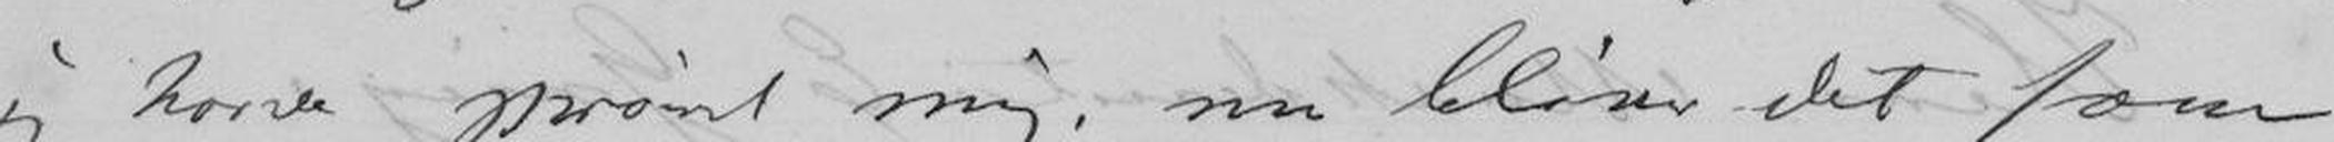jeg havde prøvet mig, nu bliver det som
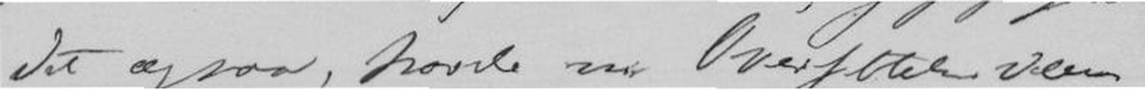det ogsaa, havde nu Oversettelse var
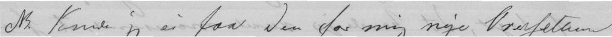NB. Kunde jeg ei faa den for mig nye Oversetteren
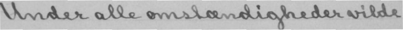Under alle omstændigheder vilde
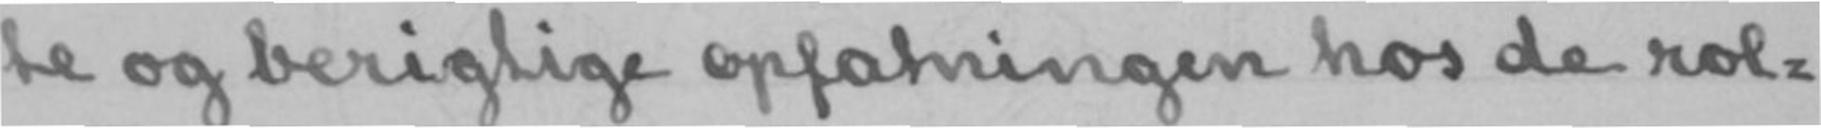te og berigtige opfatningen hos de rol-
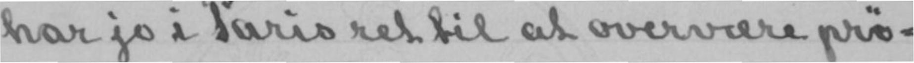har jo i Paris ret til at overvære prø-
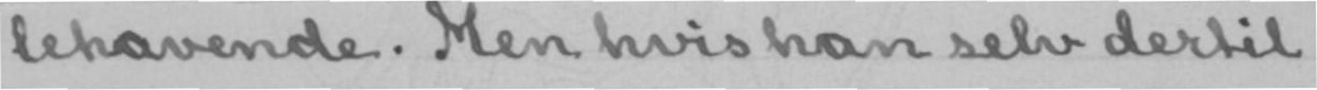lehavende. Men hvis han selv dertil
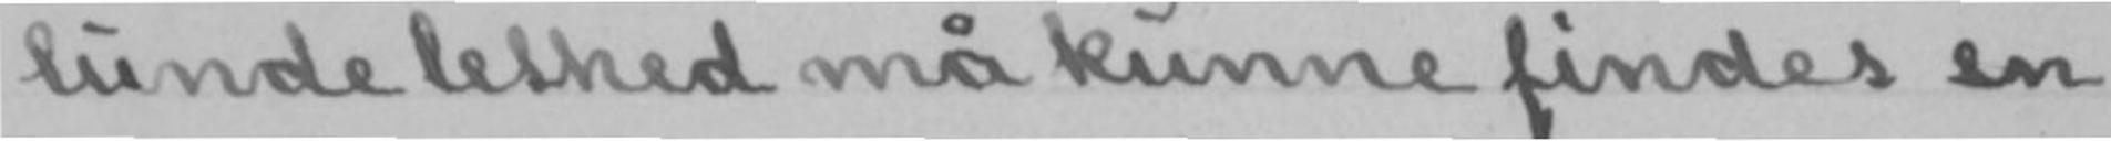lunde lethed må kunne findes en
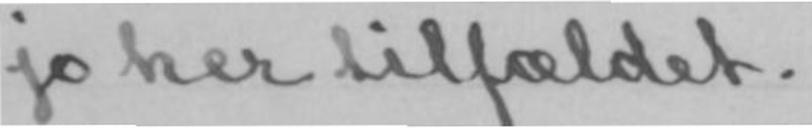jo her tilfældet.
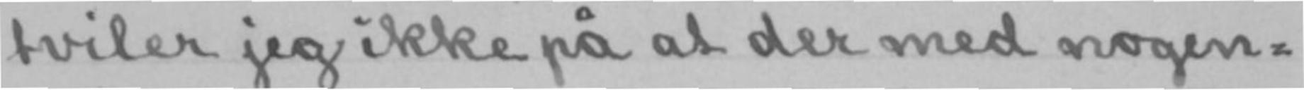tviler jeg ikke på at der med nogen-
